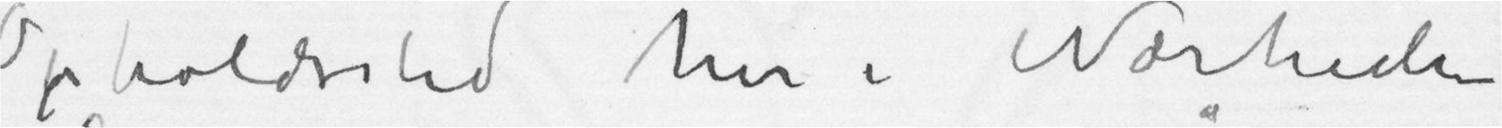Opholdssted her i Nærheden
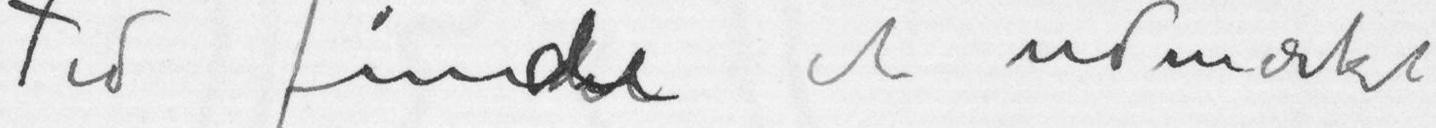Tid finde et udmærket
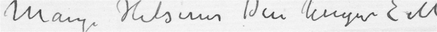Mange hilsener Din hengivne E M
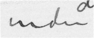under
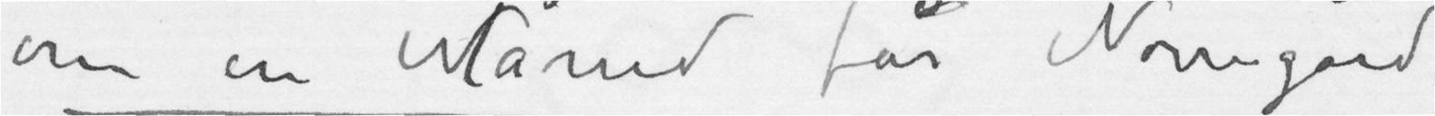om en Måned får Nørregård
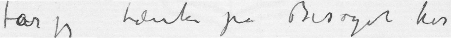får jeg tænke på Besøget hos
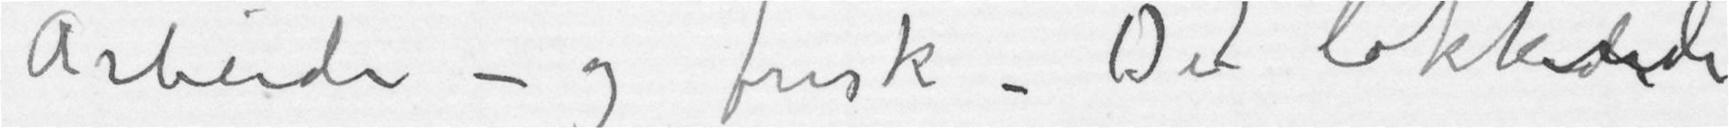Arbeide – og frisk – Det lokkende
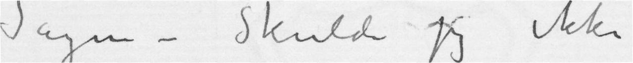Sagen – Skulde jeg ikke
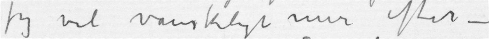jeg vel vanskeligt mer efter –
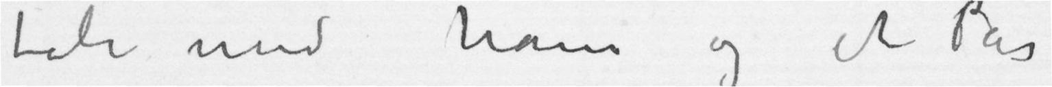tale med ham og et Par
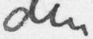den
